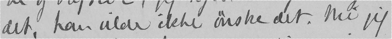det, han vilde ikke ønske det. Nei jeg
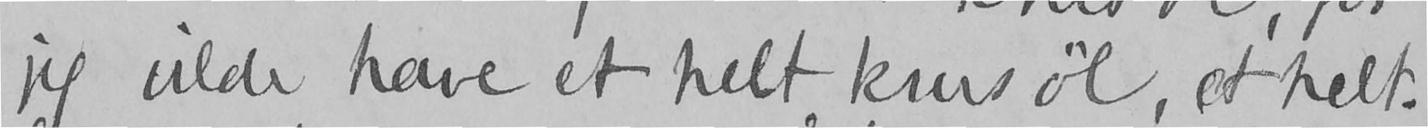jeg vilde have et helt krusøl, et helt.
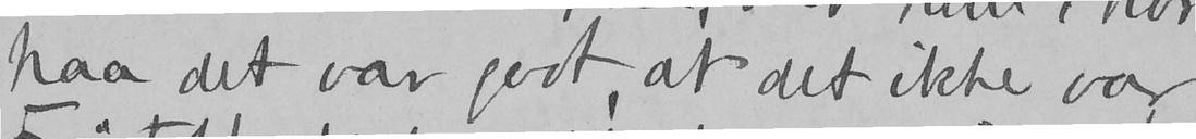haa det var godt, at det ikke var
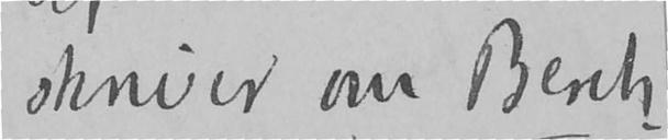skriver om Benh-
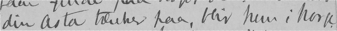din Asta tænker paa, blir hun i Norge,
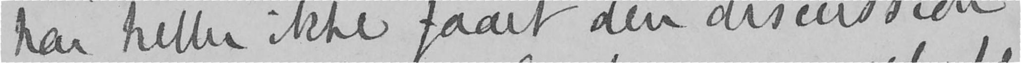har heller ikke faaet den discussion
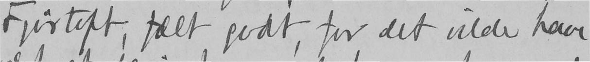Fjørtoft, fælt godt, for det vilde have
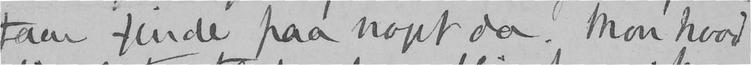faar finde paa noget da. Mon hvad
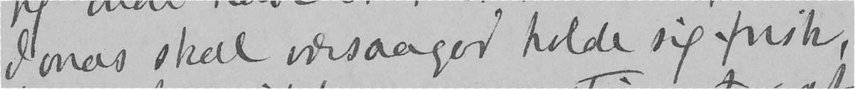Jonas skal værsaagod holde sig frisk,
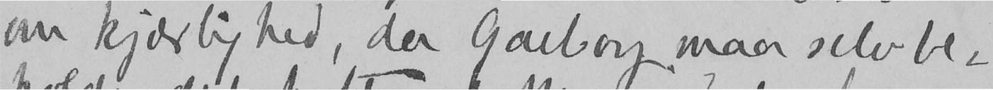om kjærlighed, da Garborg maa selv be-
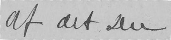af det Du
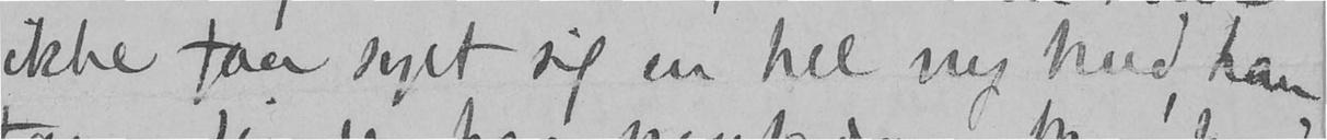ikke faa syet sig en hel ny hud, han
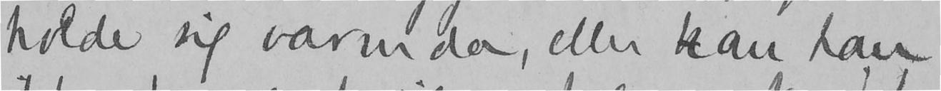holde sig varm da, eller kan han
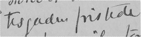tesgaden fristede
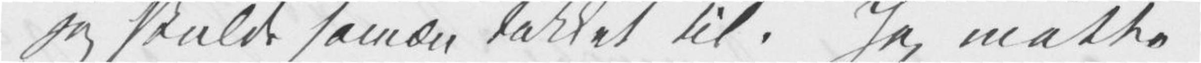jeg skulde såmæn takket til. Jeg måtte
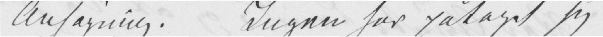Ansøgning. Ingen har påtaget sig
